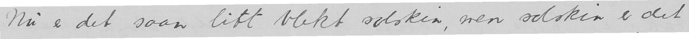Nu er det saan litt blekt solskin, men solskin er det
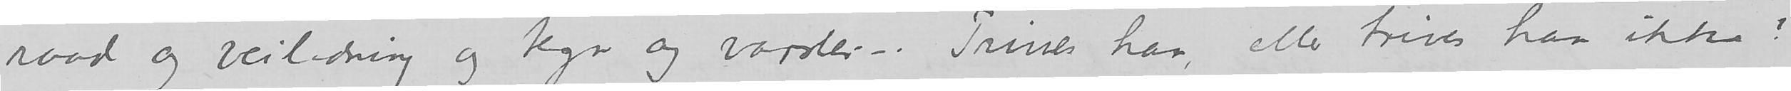raad og veiledning og tegn og varsler –. Trives han eller trives han ikke?
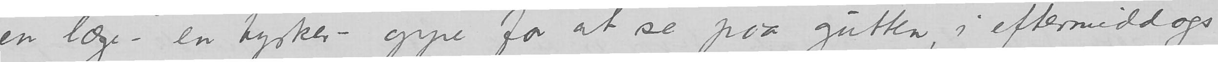en læge – en tysker – oppe for at se paa gutten, i eftermiddags
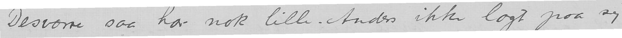Desværre saa har nok lille Anders ikke lagt paa sig
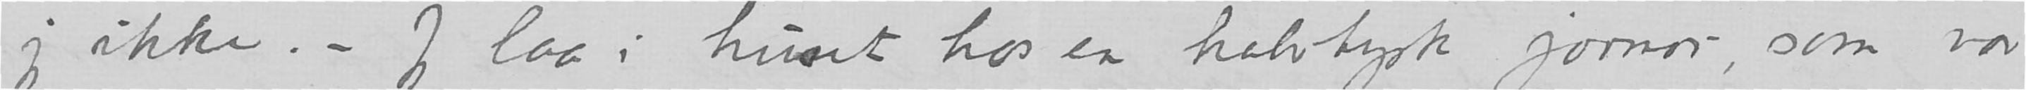jeg ikke. – Jeg laa i huset hos en halvtysk jormor, som var
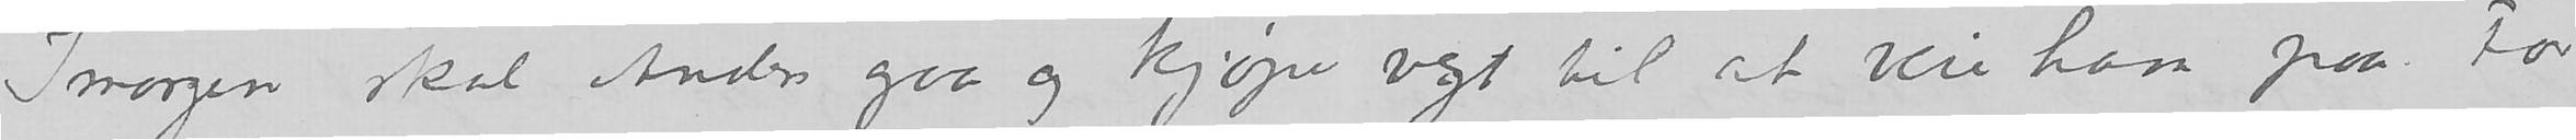Imorgen skal Anders gaa og kjøpe vegt til at veie ham paa. For
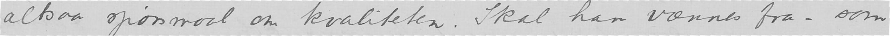altsaa spørsmaal om kvaliteten. Skal han vænnes fra – som
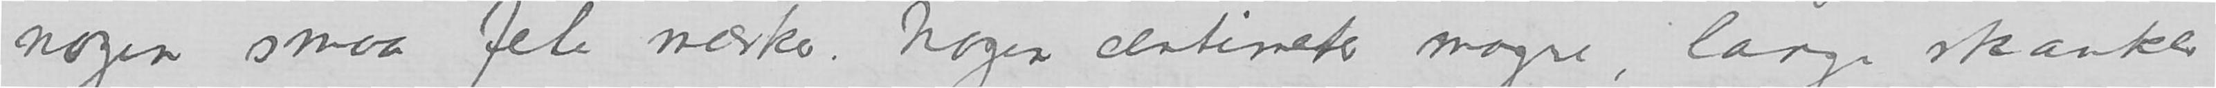nogen smaa fete merker. Nogen centimeter magre, lange skanker
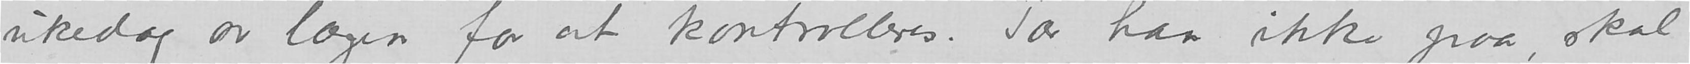ukedag av lægen for at kontrolleres. Tar han ikke paa, skal
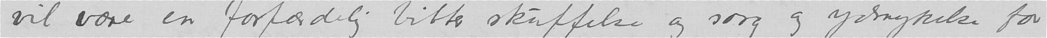vil være en forfærdelig bitter skuffelse og sorg og ydmykelse for
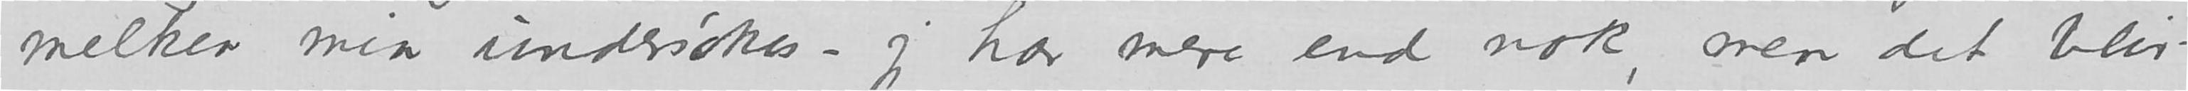melken min undersøkes – jeg har mere end nok, men det blir
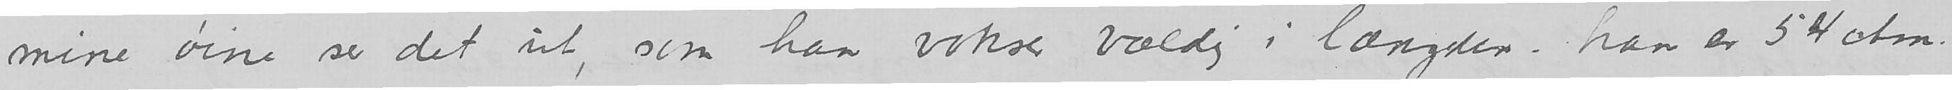mine øine ser det ut, som han vokser vældig i længden – han er 54 ctm.
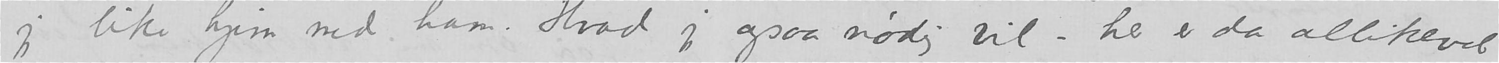jeg like hjem med ham. Hvad jeg ogsaa nødig vil – her er da allikevel
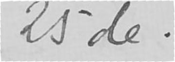25de.
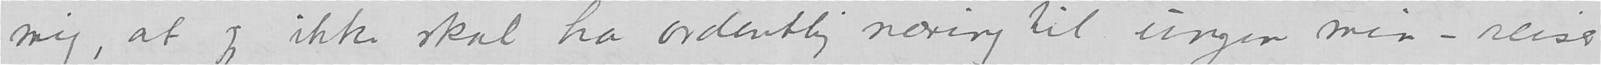mig, at jeg ikke skal ha ordentlig næring til ungen min – reiser
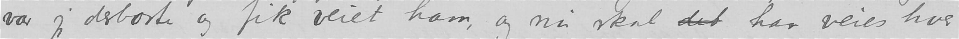var jeg derborte og fik veiet ham, og nu skal det han veies hver
